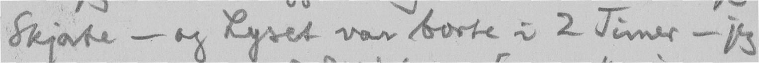Skjorte - og Lyset var borte i 2 Timer - jeg
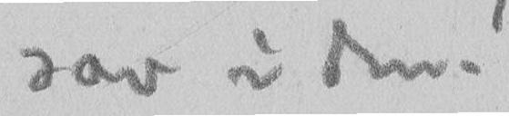sov i den.
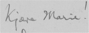Kjære Marie!
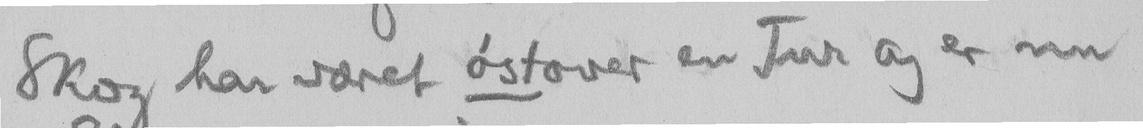Skog har været østover en Tur og er nu
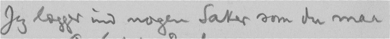Jeg lægger ind nogen Saker som du maa
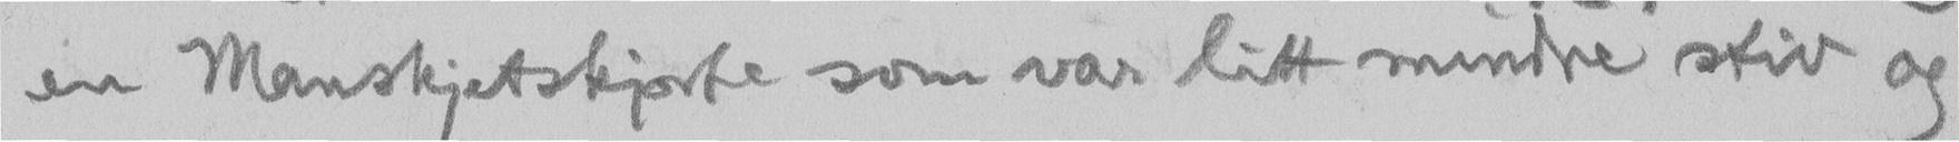en Manskjetskjorte som var litt mindre stiv og
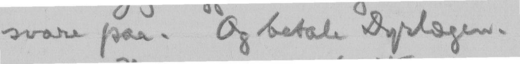svare paa. Og betale Dyrlægen.
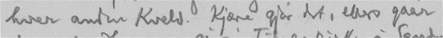hver anden Kveld. Kjære gjør det, ellers gaar
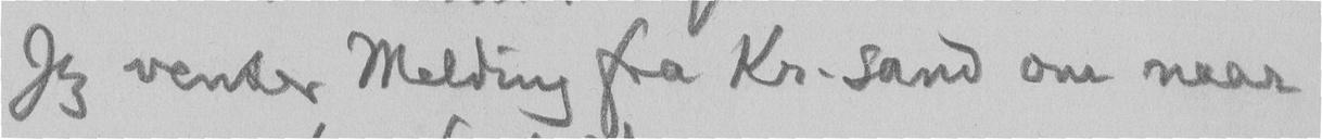Jeg venter Melding fra Kr.sand om naar
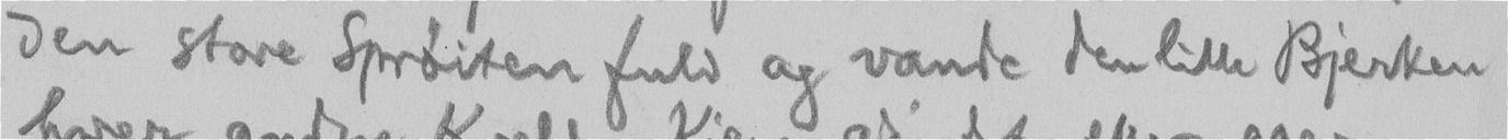den store Sprøiten fuld og vande den lille Bjerken
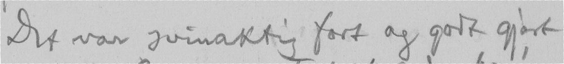Det var svinaktig fort og godt gjort
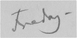Fredag.
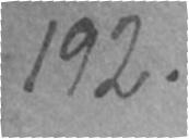192.
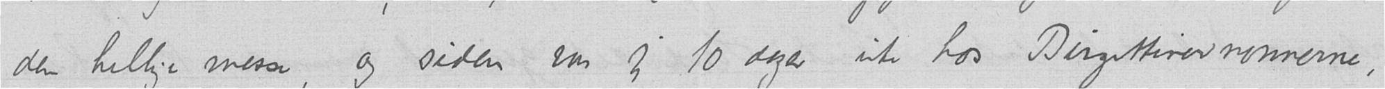den hellige messe, og siden var jeg 10 dager ute hos Birgittinernonnerne,
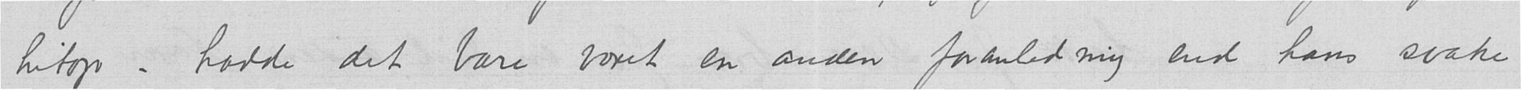hitop – hadde det bare været en anden foranledning end hans svake
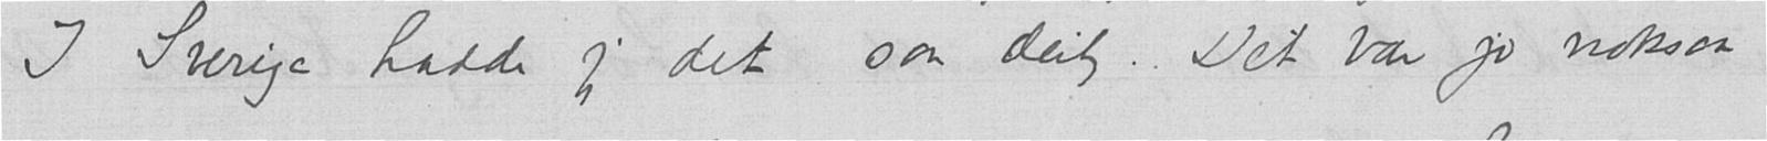I Sverige hadde jeg det saa deilig. Det var jo noksaa
